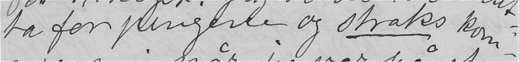ta for pengene og straks kom-
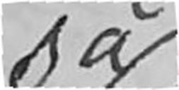så
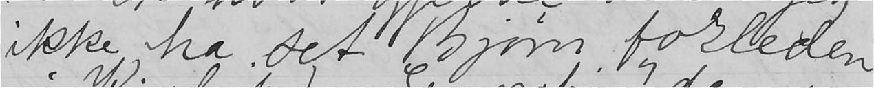ikke ha sét Bjørn forleden
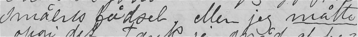småens fødsel. Men jeg måtte
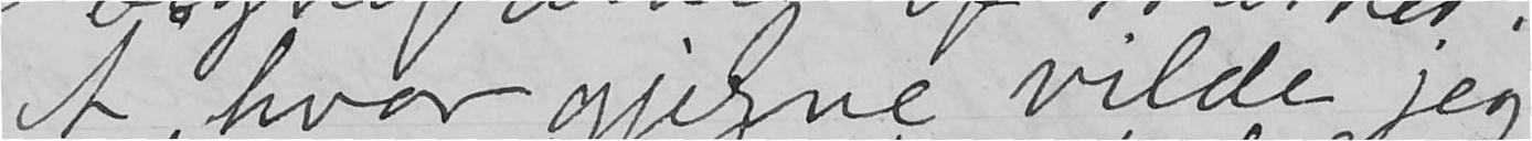Å, hvor gjerne vilde jeg
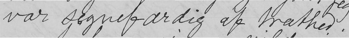var segnefærdig af træthed.
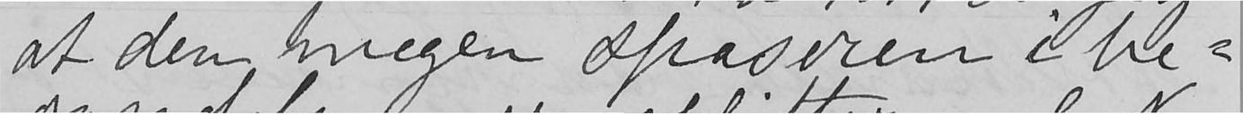at den megen spaseren i be-
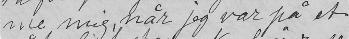me mig, når jeg var på et
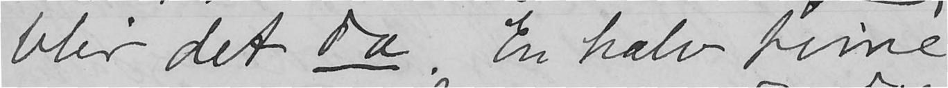blir det da. En halv time
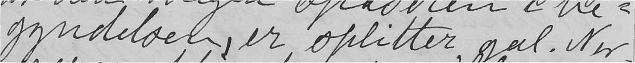gyndelsen, er splitter gal. Ner-
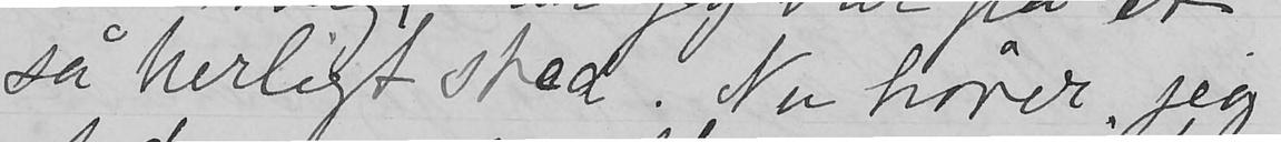så herligt sted. Nu hører jeg
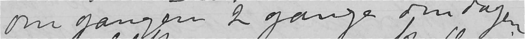om gangen 2 gange om dagen
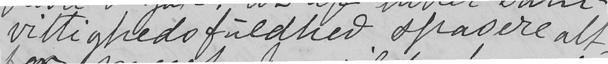vittighedsfuldhed spasere alt-
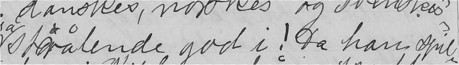strålende god i! Da han spil-
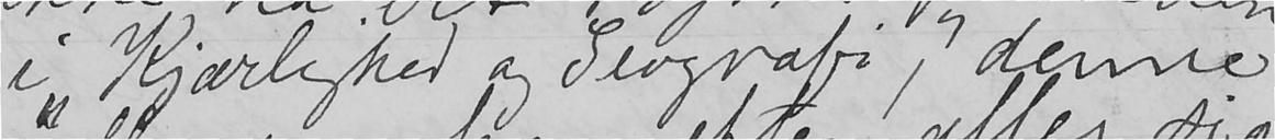i "Kjærlighed og Geografi", denne
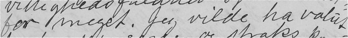for meget. Jeg vilde ha valut-
